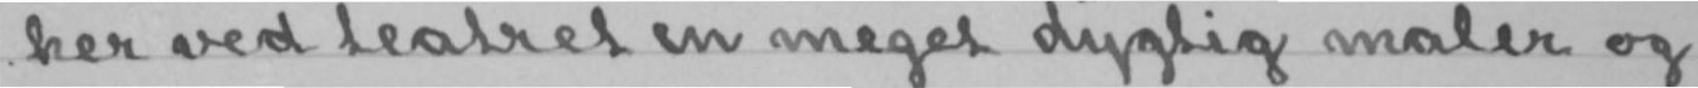her ved teatret en meget dygtig maler og
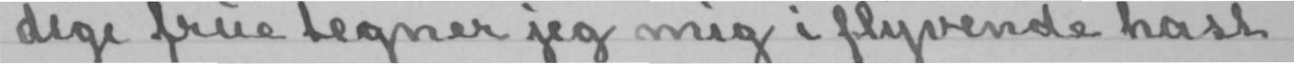dige frue tegner jeg mig i flyvende hast
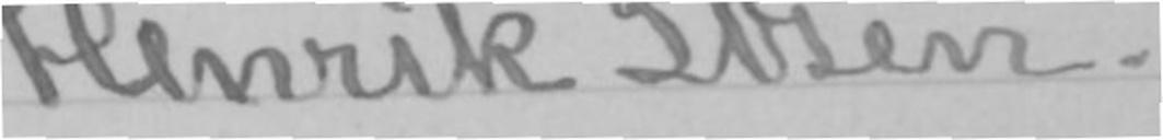Henrik Ibsen.
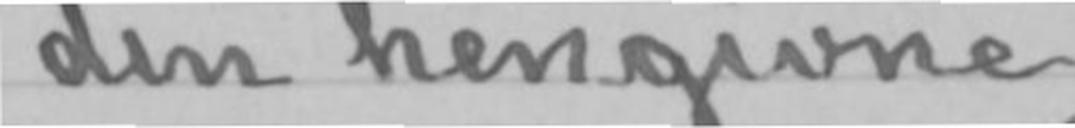din hengivne
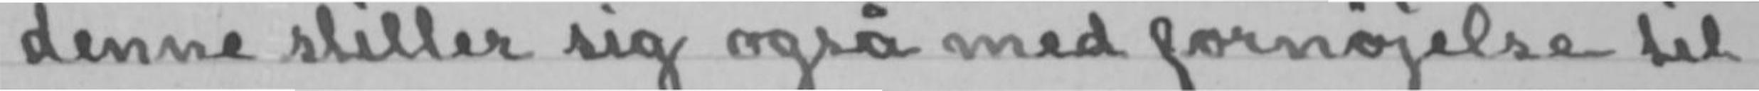denne stiller sig også med fornøjelse til
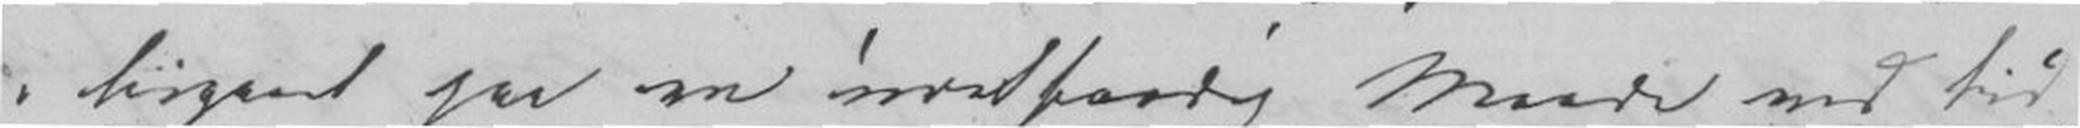bigaaet paa en uretfærdig Maade ved tid
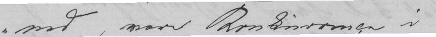ned, være Konkurrance i
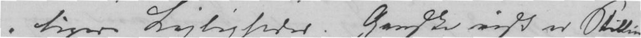ligere Lejligheder. Ganske viss er Stillin-
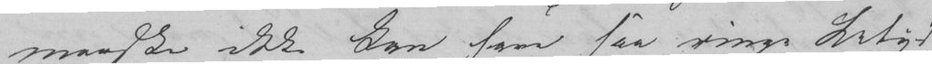maaske ikke kan have saa ringe Betyd-
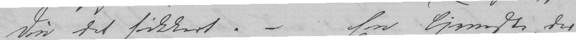Du det sikkert. - En tjeneste, der
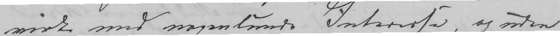møde med nogenlunde Interesse, og uden
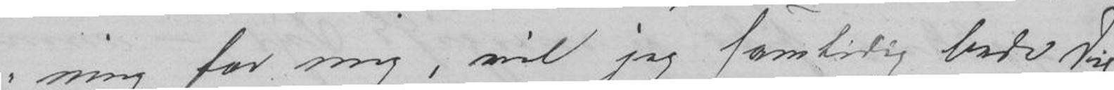ning for mig, vil jeg samtidig bede Dig
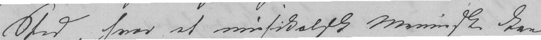sted, hvad et musikalsk Menneske kan
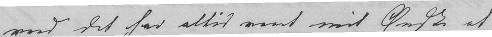ved det har alltid været mit Ønske at
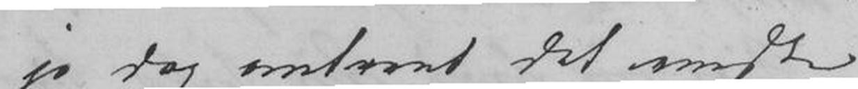jo dog omtrent det eneste
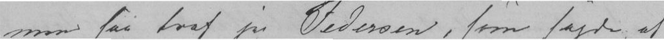men saa traf jeg Federsen, som sagde; at
